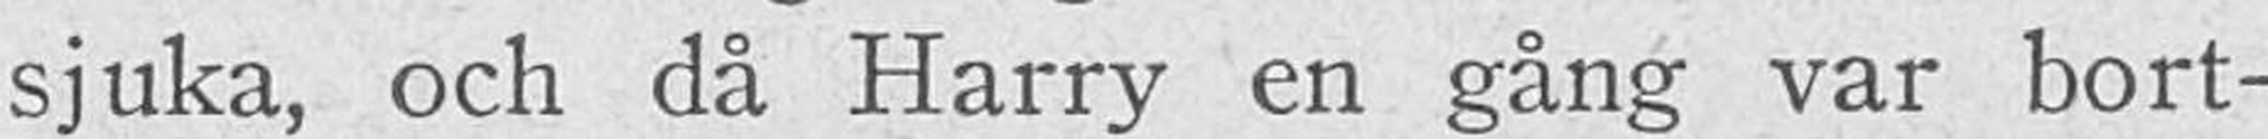sjuka, och då Harry en gang var bort-
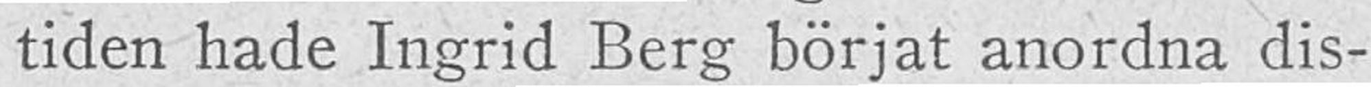tiden hade Ingrid Berg börjat anordna dis-
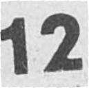12
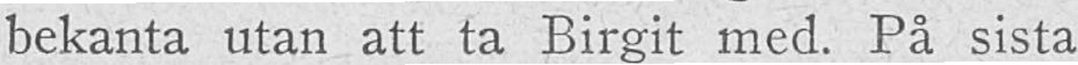bekanta utan att ta Birgit med. På sista
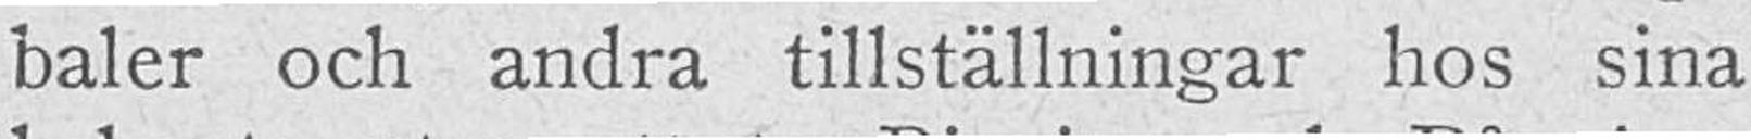baler och andra tillställningar hos sina
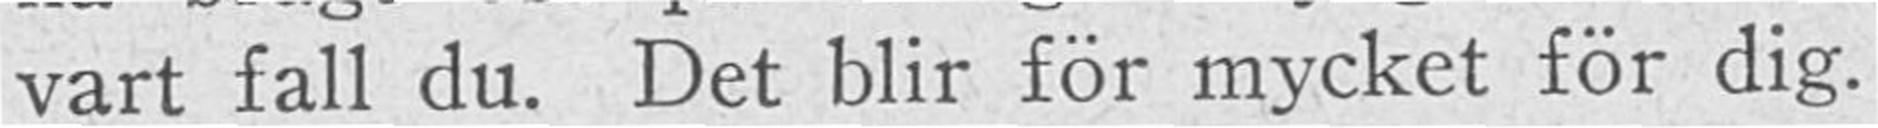vart fall du. Det blir för mycket för dig.
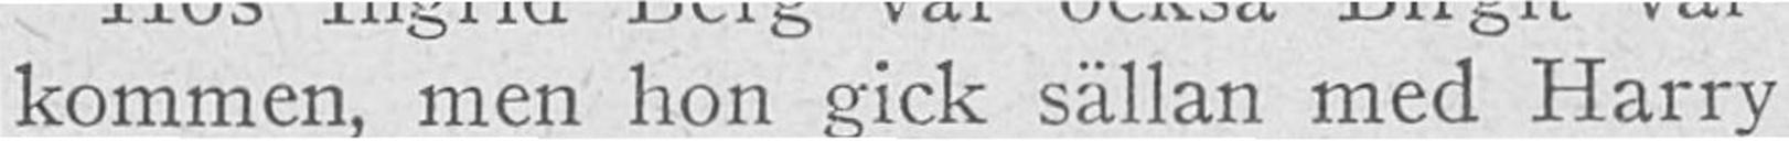kommen, men hon gick sällan med Harry
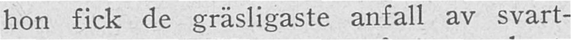hon fick de gräsligaste anfall av svart-
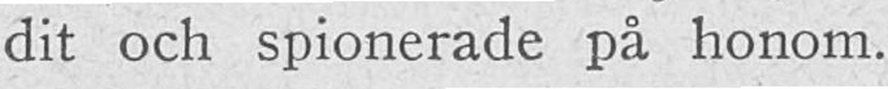dit och spionerade på honom.
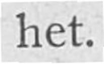het.
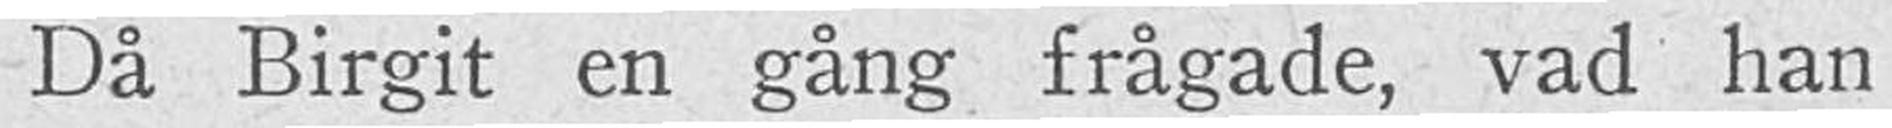Då Birgit en gång frågade, vad han
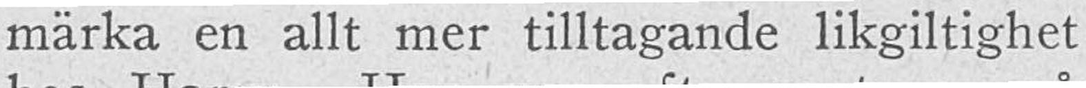märka en allt mer tilltagande likgiltighet
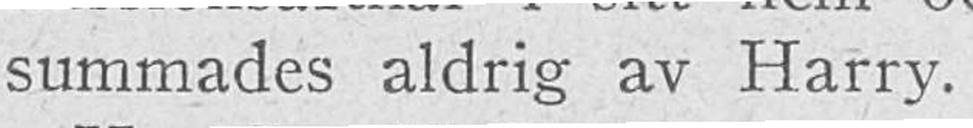summades aldrig av Harry.
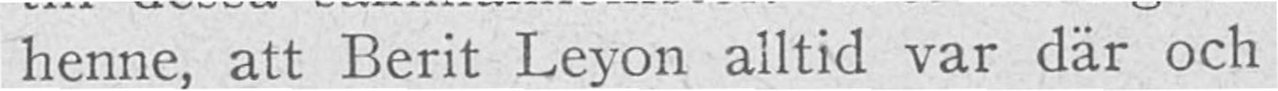henne, att Berit Leyon alltid var där och
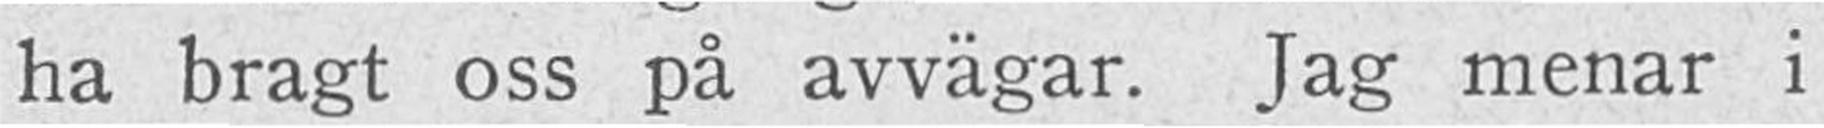ha bragt oss på avvägar. Jag menar i
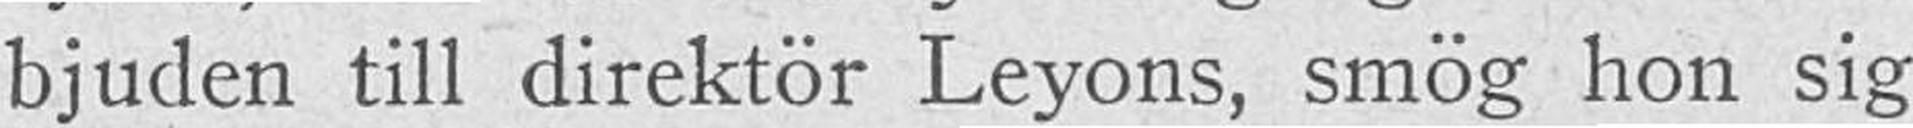bjuden till direktör Leyons, smög hon sig
